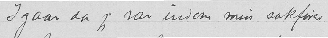I gaar da jeg var indom min sakfører.
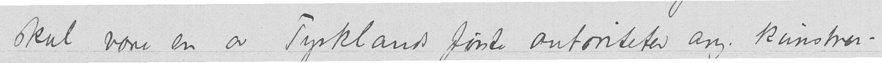skal være en av Tysklands første antoriteter ang. kanstner-
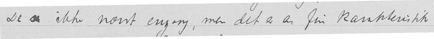De ser ikke nævnt engang, men det er en fin karakteristik
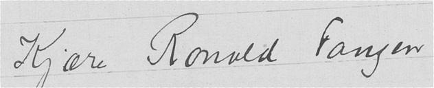Kjære Ronald Fangen
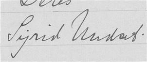Sigrid Undset.
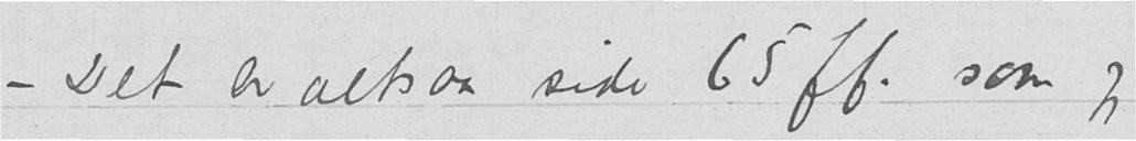– Det er altsaa side 65 ff. som jeg
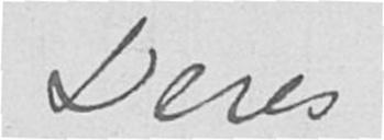Deres
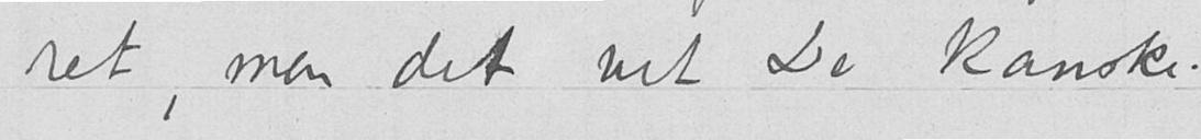ret, men det vet De kanske.
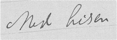Med hilsen
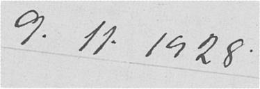9.11.1928.
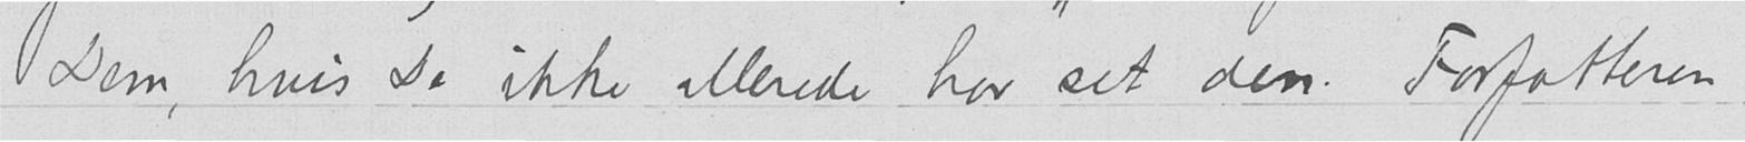Dem, hvis De ikke allerede har set den. Forfatteren
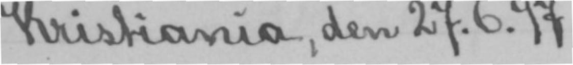Kristiania, den 27.6.97.
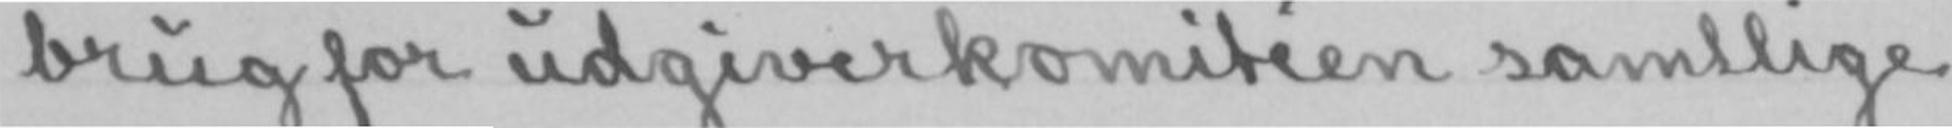brug for udgiverkomitéen samtlige
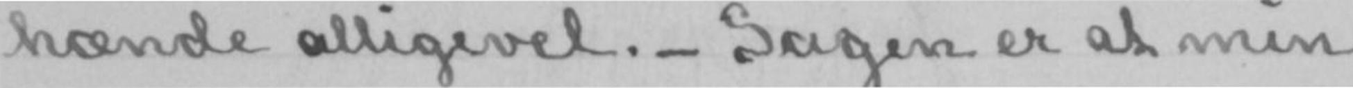hænde alligevel.- Sagen er at min
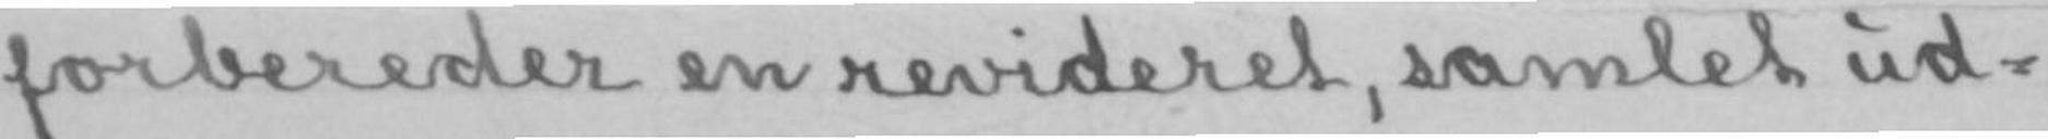forbereder en revideret, samlet ud-
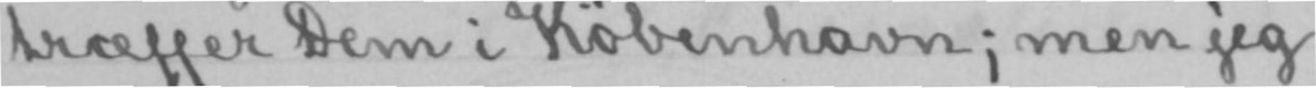træffer Dem i København; men jeg
